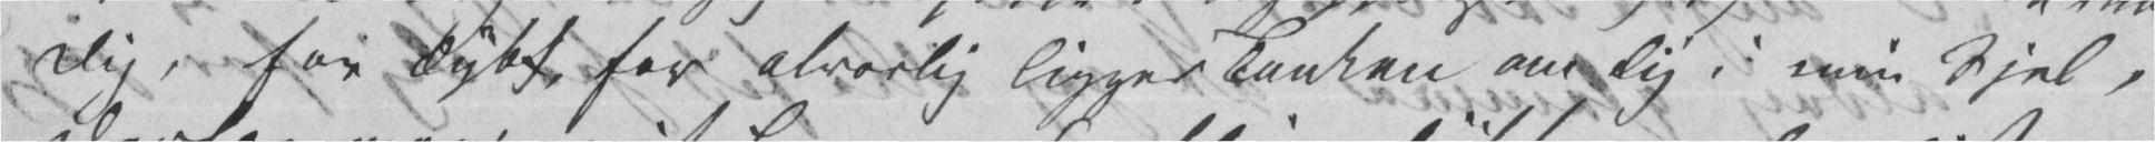Dig, for dybt, for alvorlig ligger Tanken om Dig i min Sjel,
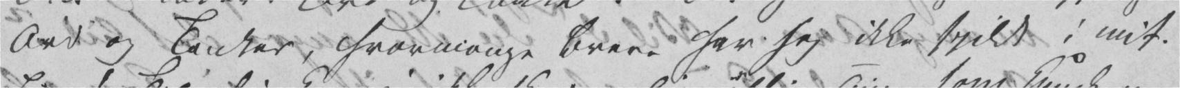Ord og Tanker, hvormange Breve har jeg ikke spildt i mit
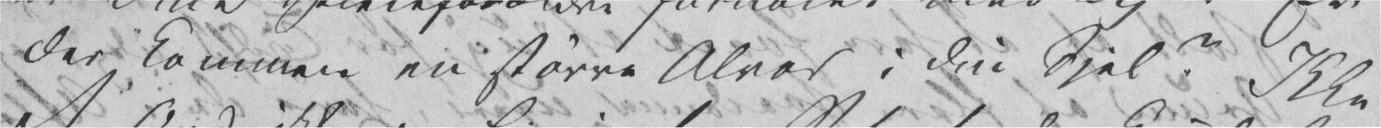der kommen en større Alvor i din Sjel? Ikke
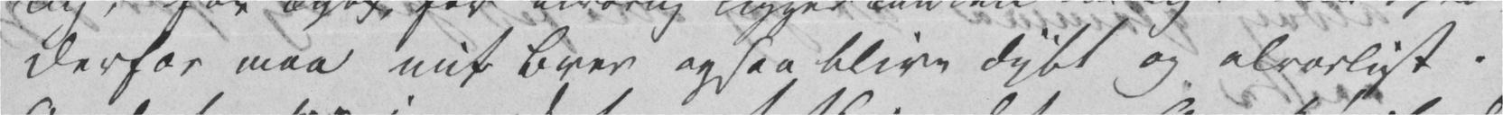derfor maa mit Brev ogsaa blive dybt og alvorligt.
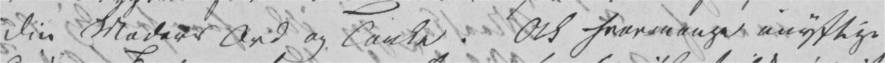Din Moders Ord og Tanke. Ak hvormange unyttige
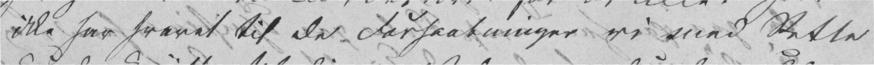ikke har svaret til de Forhaabninger vi med Rette
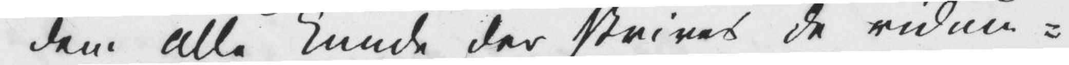dem alle kunde der skrives de vidun¬
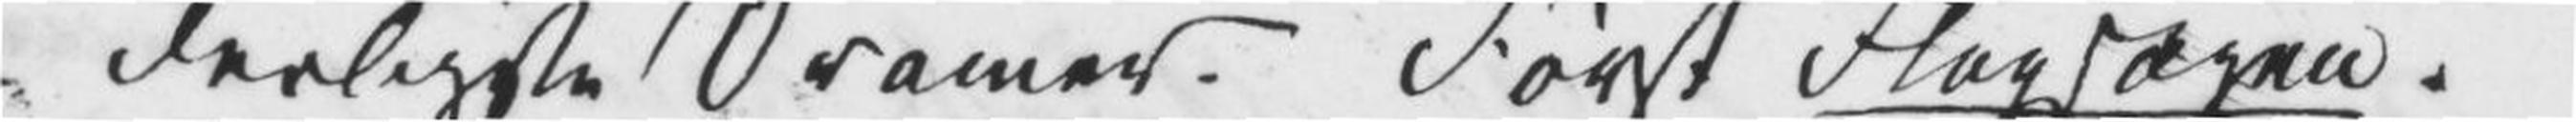derligste Dramer. Først Flagsagen.
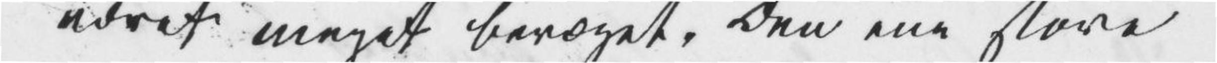været meget bevæget. Den ene store
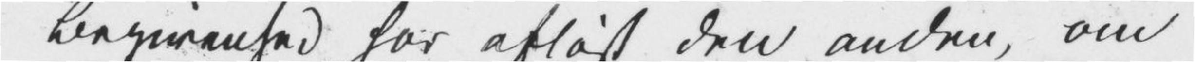Begivenhed har afløst den anden, om
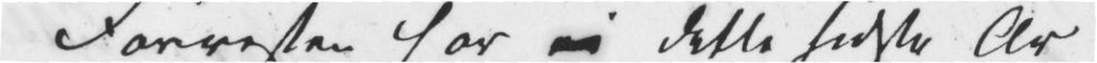Forresten har dette sidste År
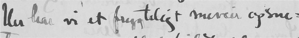Her har vi et frygteligt sneveir og sne-
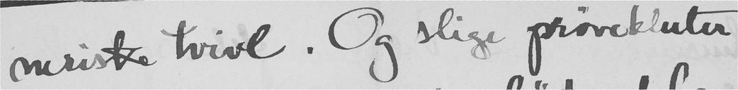neriske tvivl. Og slige prøvekluter
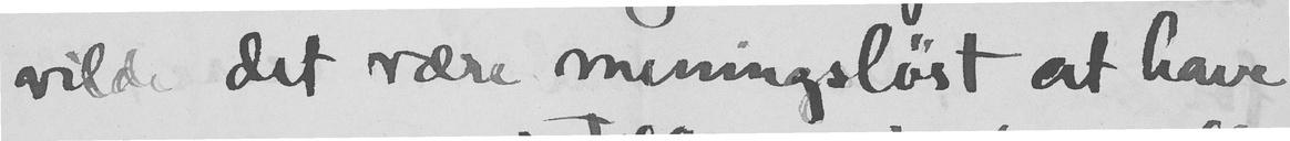vilde det være meningsløst at have
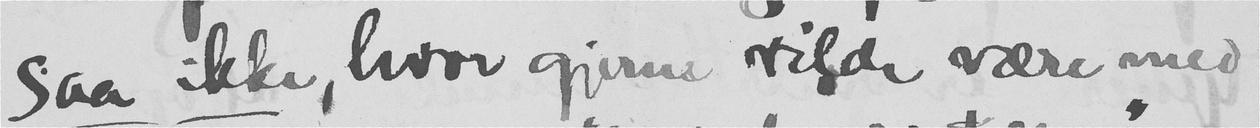saa ikke, hvor gjerne vilde være med
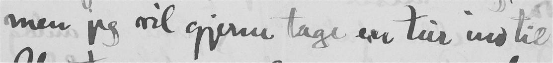men jeg vil gjerne tage en tur ind til
